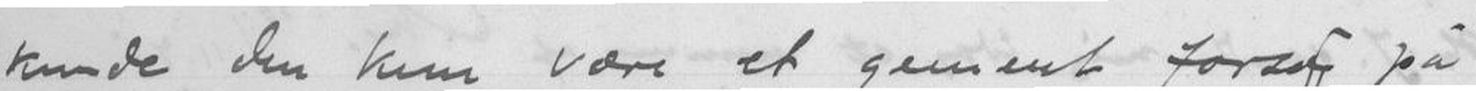kunde den kun være et gement forsøg på
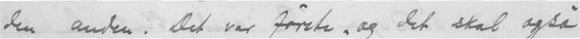den anden. Det var første, og det skal også
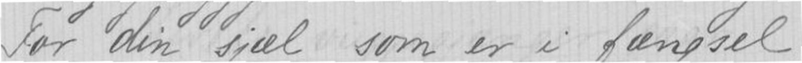For din sjæl som er i fængsel,
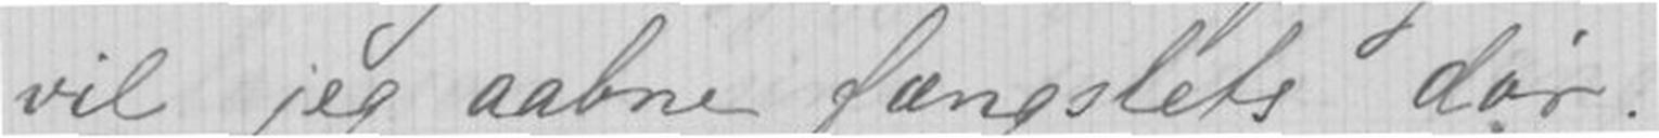vil jeg aabne fængslets dør.
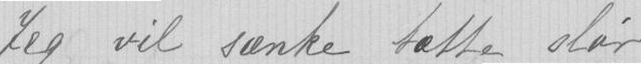Jeg vil sænke tætte slør
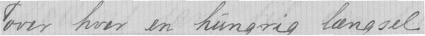over hver en hungrig længsel,
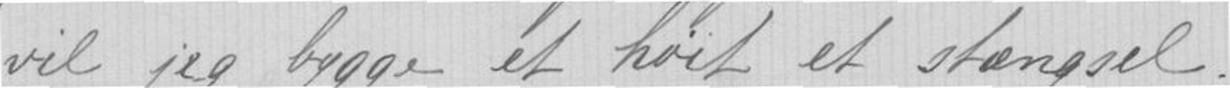vil jeg bygge et høit et stængsel.
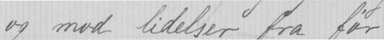og mod lidelser fra før
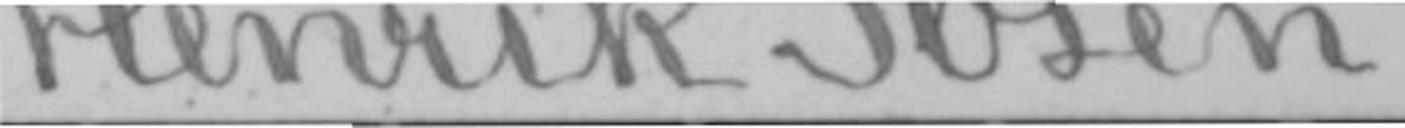Henrik Ibsen.
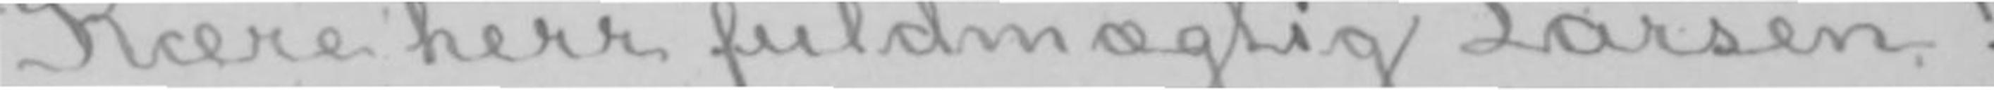Kære herr fuldmægtig Larsen!
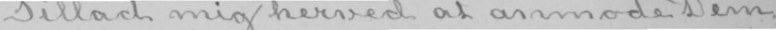Tillad mig herved at anmode Dem
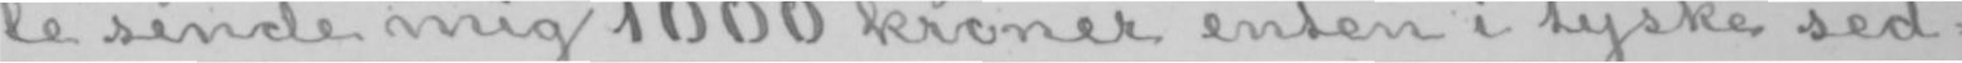le sende mig 1000 kroner enten i tyske sed-
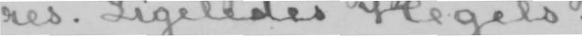res. Ligeledes Hegels.
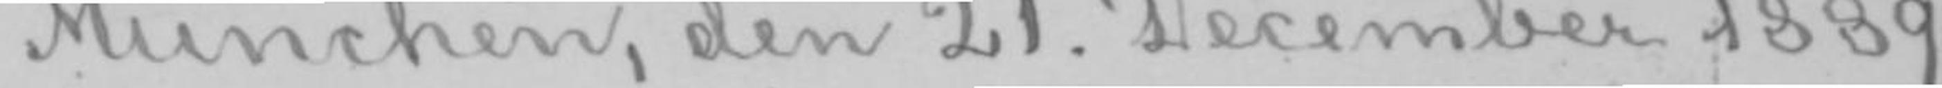München, den 21. December 1889.
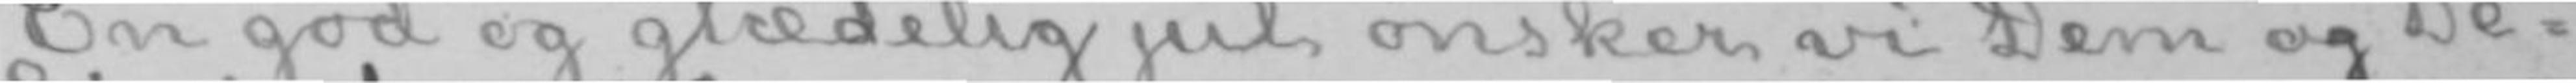En god og glædelig jul ønsker vi Dem og De-
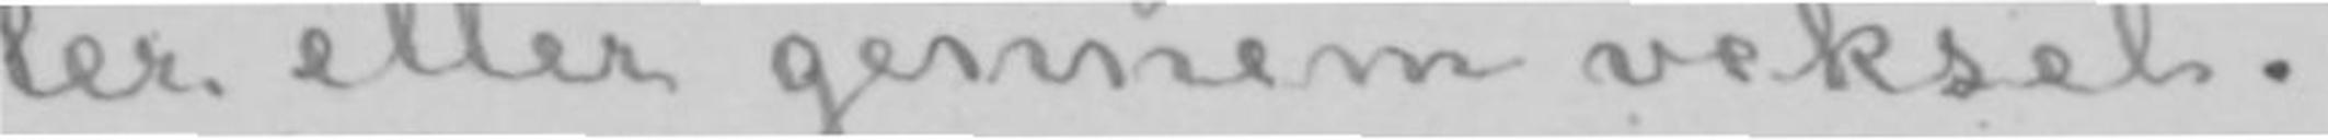ler eller gennem veksel.
